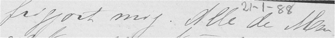frigjort mig. Alle de Men-
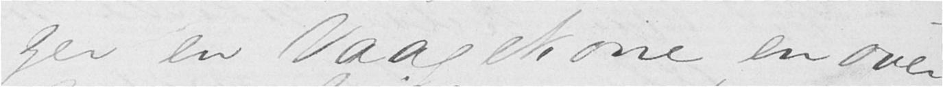ger en Vaagekone en over-
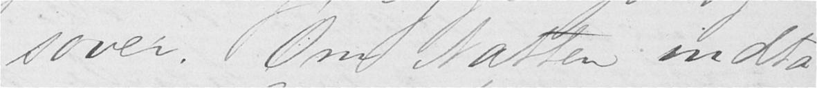sover. Om Natten indta-
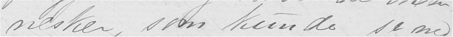nesker, som kunde se ned
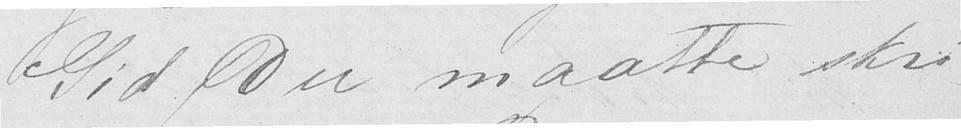Gid Du maatte skri-
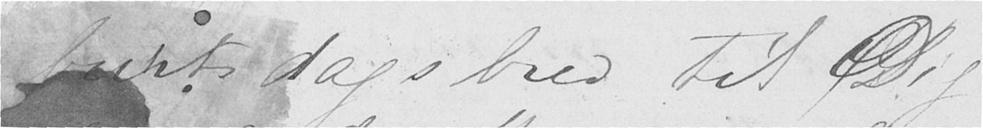burtsdagsbrev til Dig
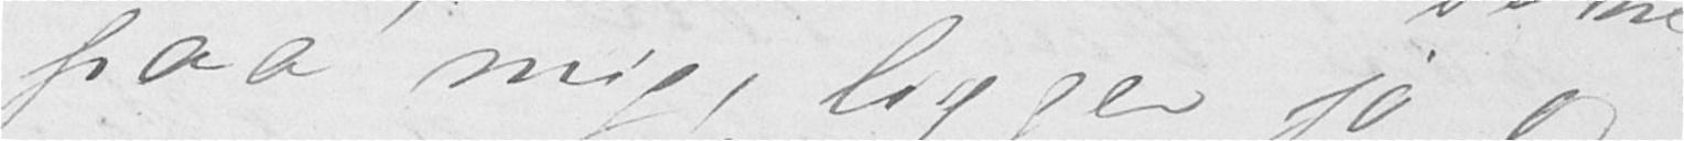paa mig, ligger jo og
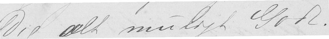Dig alt muligt Godt.
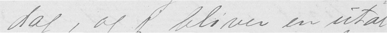dag, og jeg bliver en utal-
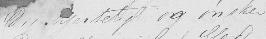Dig hjerteligt og ønsker
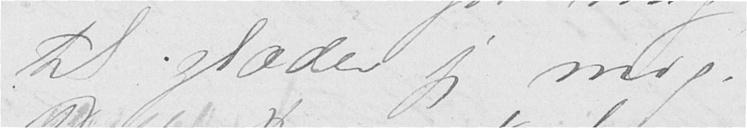til glæder jeg mig.
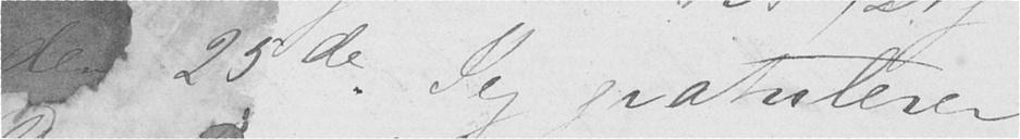de 25de. Jeg gratulerer
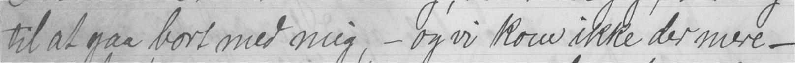til at gaa bort med mig, - og vi kom ikke der mere -
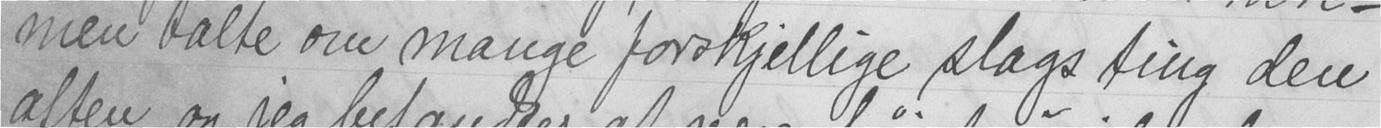men talte om mange forskjellige slags ting den
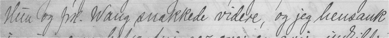Hun og frk. Wang snakkede videre, og jeg hensank
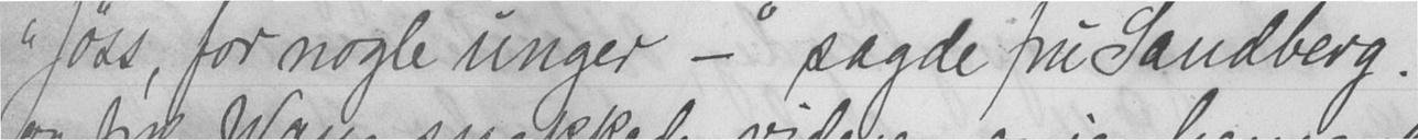"Jøss, for nogle unger - " sagde fru Sandberg.
